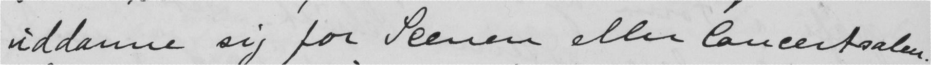uddanne sig for Scenen eller Concertsalen.
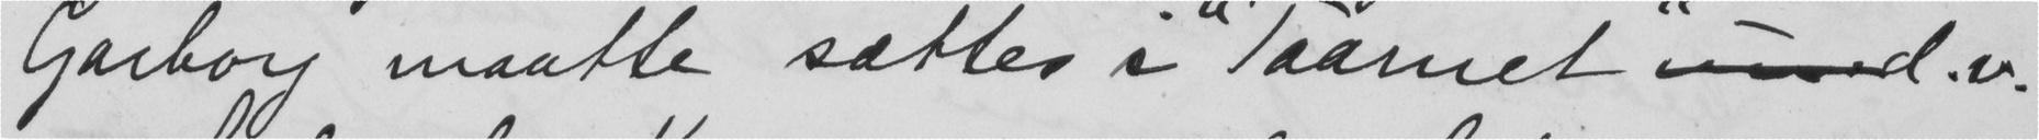Garborg maatte sættes i "Taarnet"  d.v.
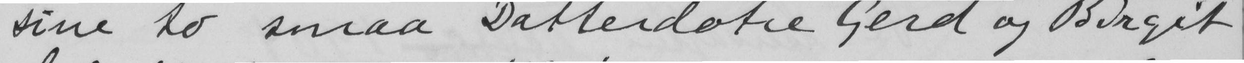sine to smaa Datterdøtre Gerd og Birgit
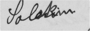Solskin
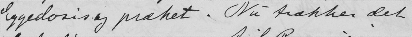Eggedosis og præket. Nu trækker det
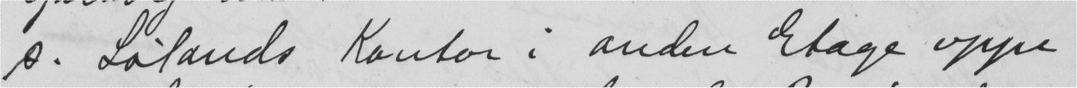s. Lølands Kontor i anden Etage oppe
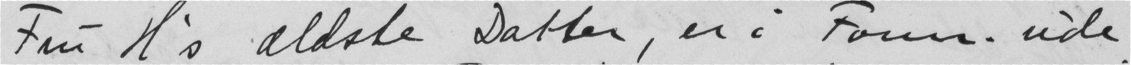Fru H's ældste Datter, er i Form. ude
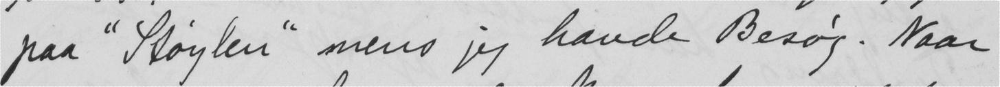paa "Støylen" mens jeg havde Besøg. Naar
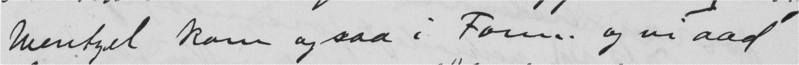Wentzel kom ogsaa i Form. og vi aad
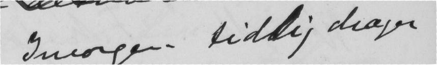Imorgen tidlig dræger
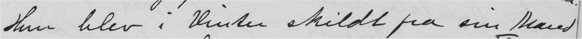Hun blev i Vinter skildt fra sin Mand
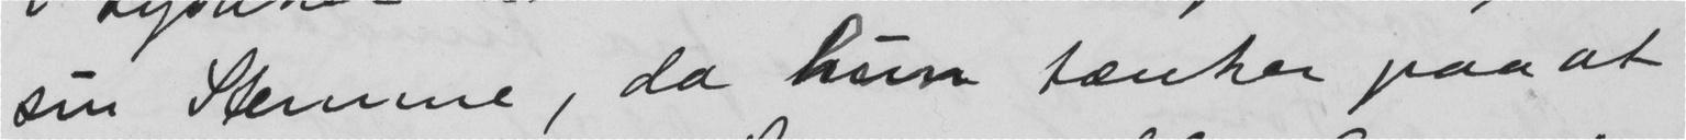sin Stemme, da hun tænker paa at
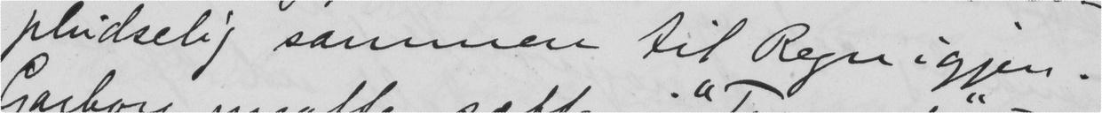pludselig sammen til Regn igjen.
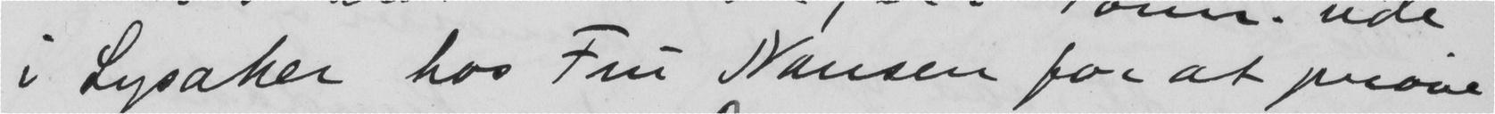i Lysaker hos Fru Nansen for at prøve
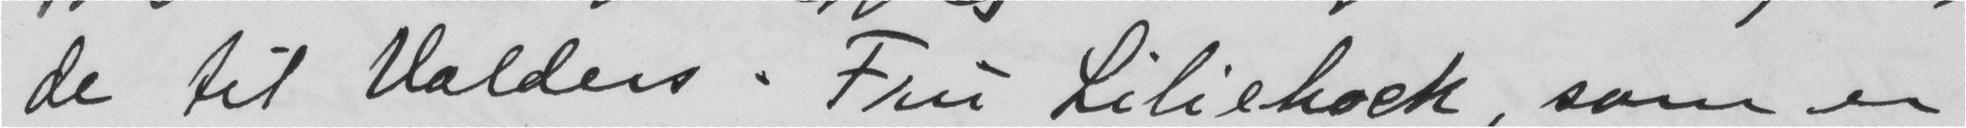de til Valders. Fru Liliehøck, som er
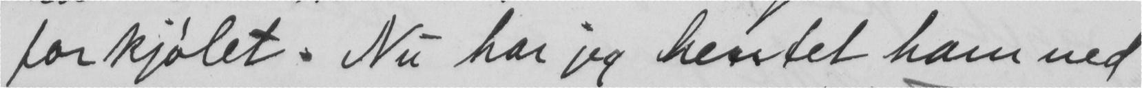forkjølet. Nu har jeg hentet ham ned
